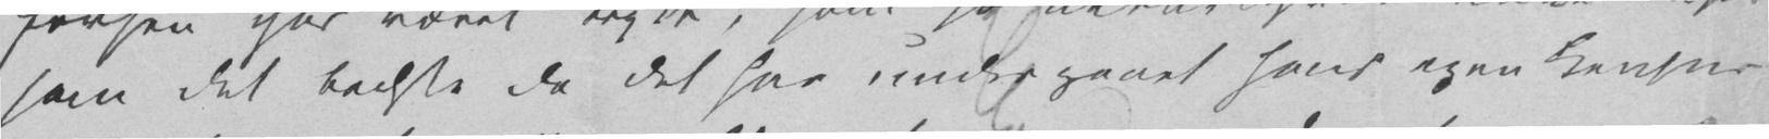som det bedste da det har undergaaet hans egen Censur
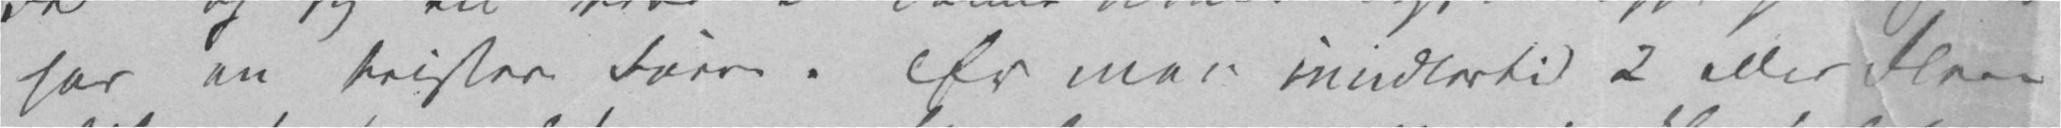har en tristere Farve. Er man imidlertid 2 eller Flere
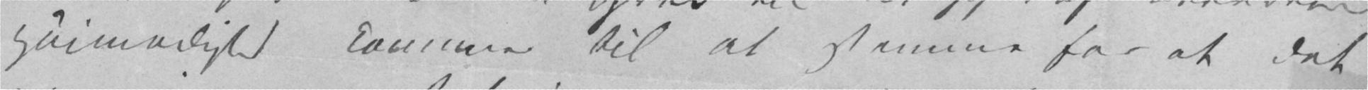Høimodighed kommer til at stemme for at det
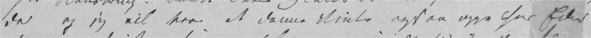de og jeg vil troe at denne Vinter ogsaa oppe hos Eder
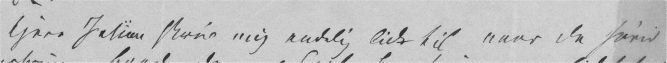Kjere Johan skriv mig endelig lidt til naar De hører
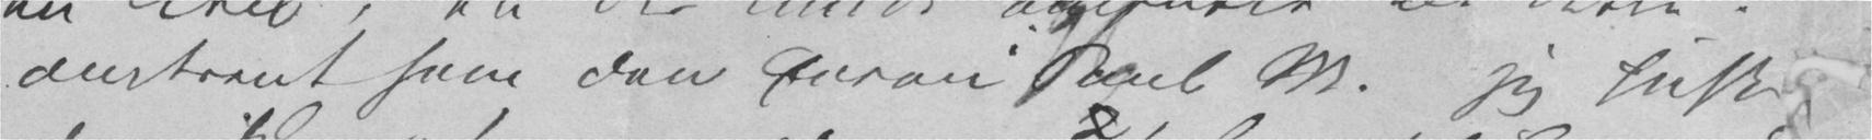omtrent som den foran i Paul M. jeg huske
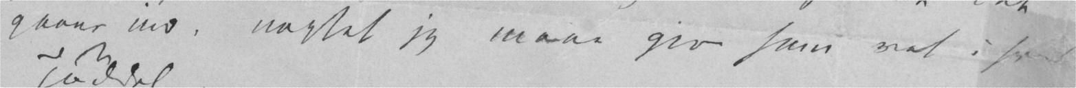gaaer ind, uagtet jeg maae give ham ret i hver
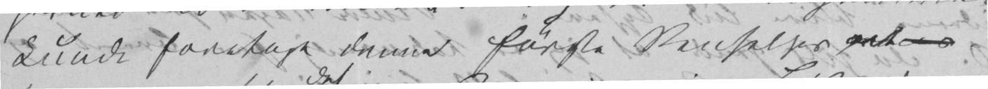kunde foretage denne første Renselsesact
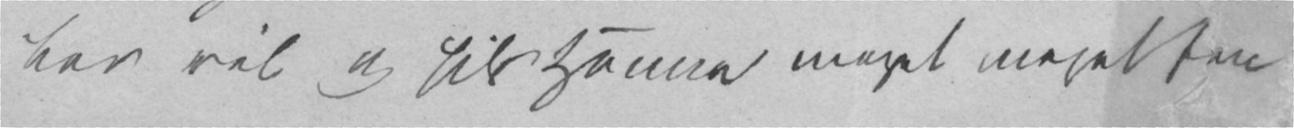Lev vel og hils Hanne meget meget fra
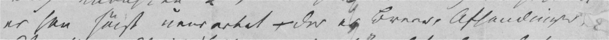er saa høist uensartet – der er Breve, Afhandlinger,
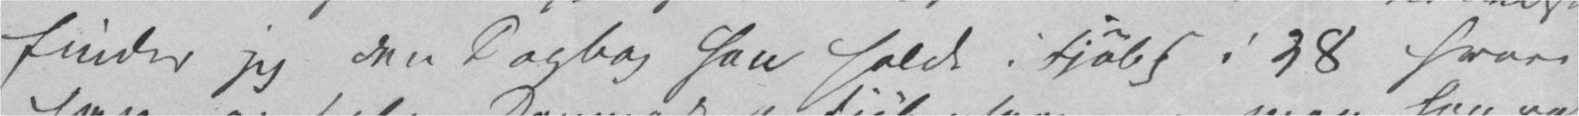finder jeg den Dagbog han holdt i Kjøbh i 38 hvori
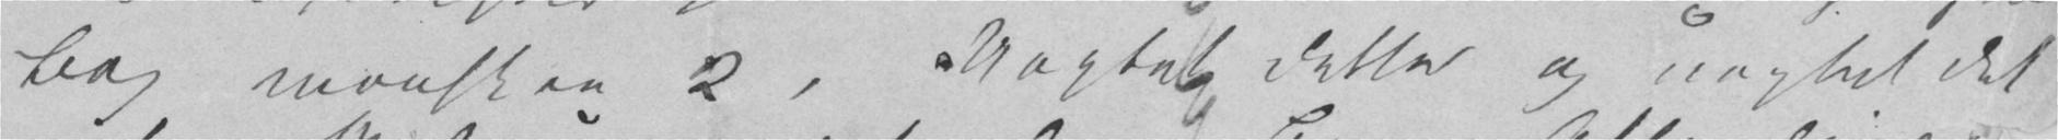Bog maaskee 2, Uagtet dette og uagtet det
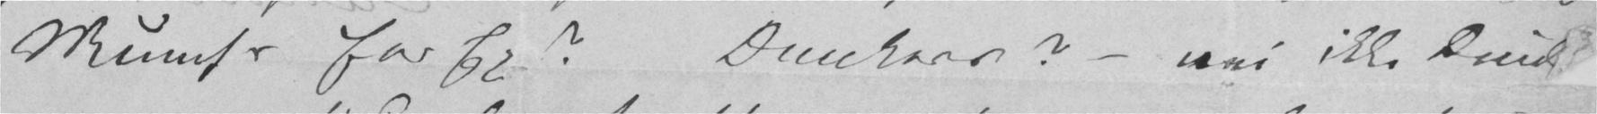Munchs for Ex? Dunkers? – nei ikke Dunker.
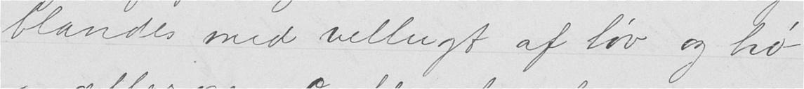blandes med vellugt af løv og hø-
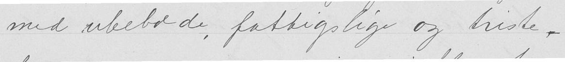med ubeboede, fattigslige og triste
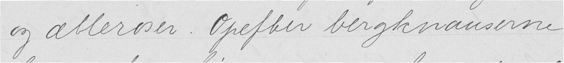og æbleroser. Opefter bergknauserne
